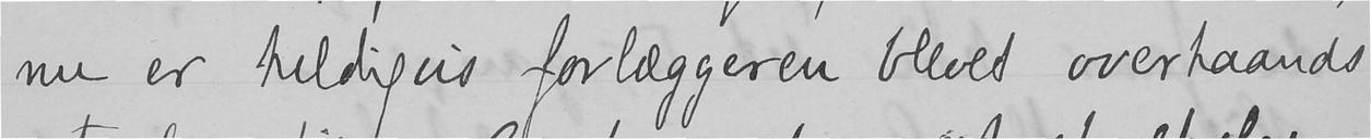nu er heldigvis forlæggeren blevet overhaands
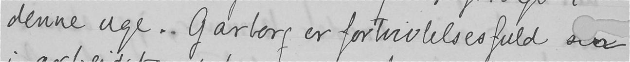denne uge. Garborg er fortvivlelsesfuld sen
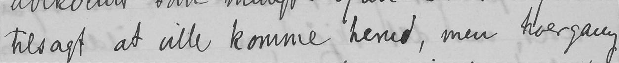tilsagt at ville komme herud, men hvergang
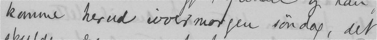komme herud iovermorgen søndag, det
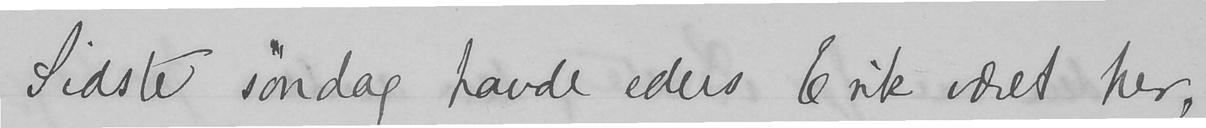Sidste søndag havde eders Erik været her,
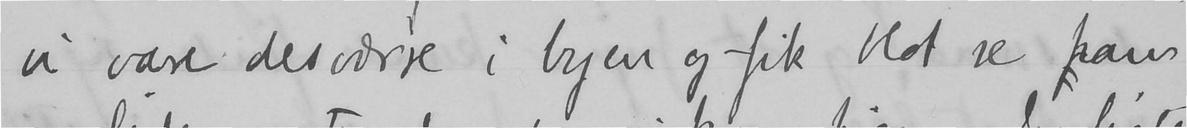vi vare desværre i byen og fik blot se ham
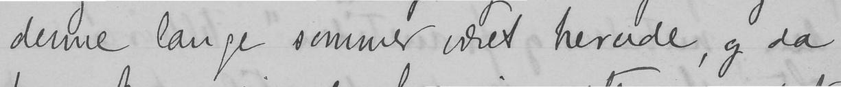denne lange sommer været herude, og da
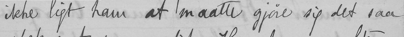ikke ligt ham at maatte gjøre sig det saa
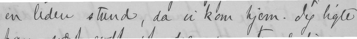en liden stund, da vi kom hjem. Jeg ligte
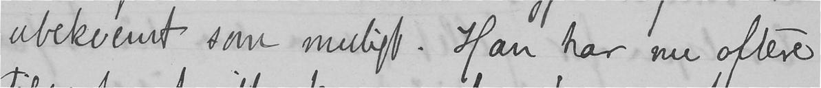ubekvemt som muligt. Han har nu oftere
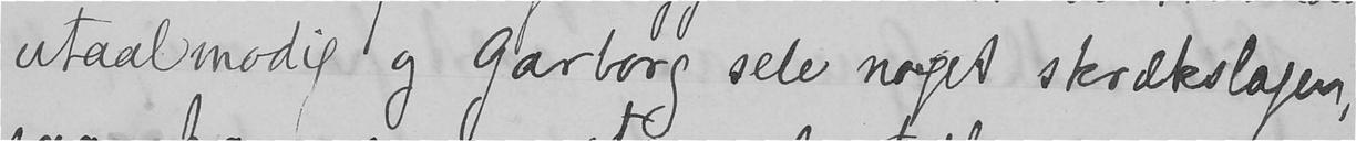utaalmodig og Garborg selv noget skrækslagen,
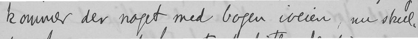kommer der noget med bogen iveien, nu skul-
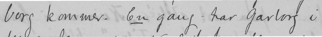borg kommer. En gang har Garborg i
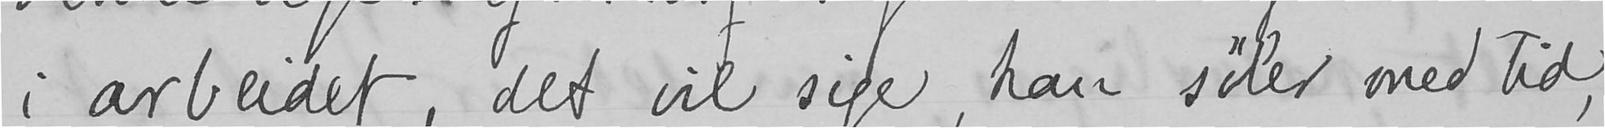i arbeidet, det vil sige, han søler med tid,
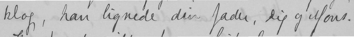klog, han lignede din fader, dig og Mons.
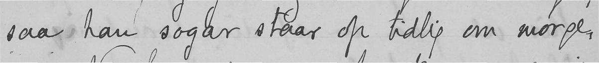saa han sogar staar op tidlig om morge-
